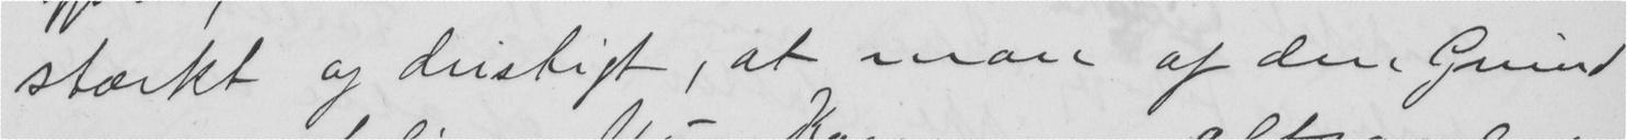stærkt og dristigt, at man af den Grund
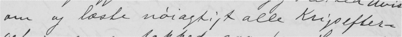om og læste nøiagtigt alle Krigsefter-
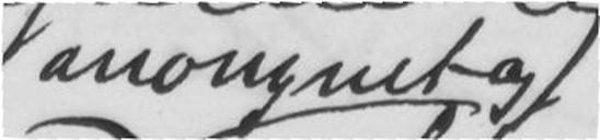anonymt og
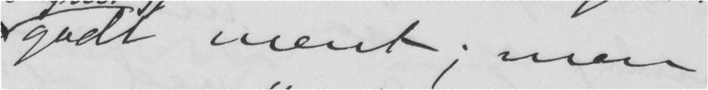godt ment; men
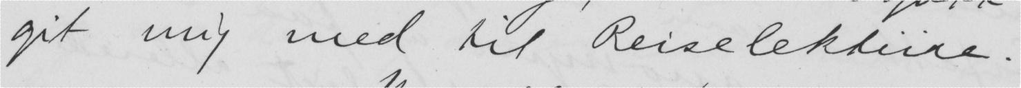git mig med til Reiselektiire.
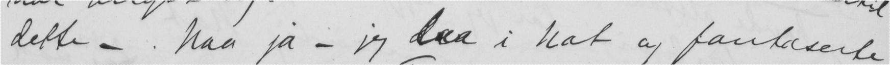dette - . Naa ja — jeg laa i Nat og fantaserte
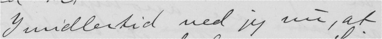Imidlertid ved jeg nu, at
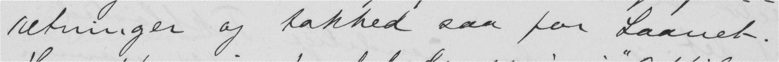retninger og takked saa for Laanet.
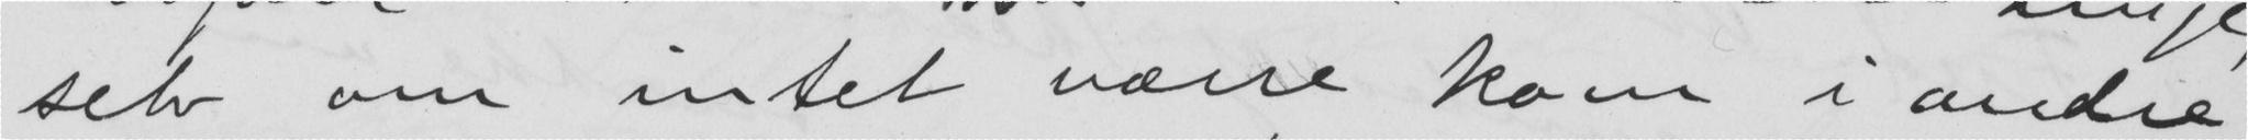selv om intet værre kom i andre
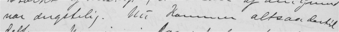var ængstelig. Nu kommer altsaa dertil
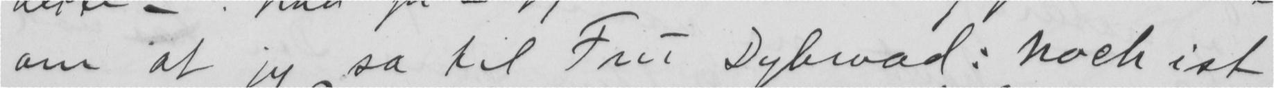om at jeg sa til Fru Dybwad: noch ist
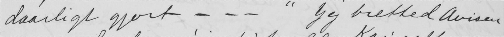daarligt gjort - - - "Jeg bretted Avisen
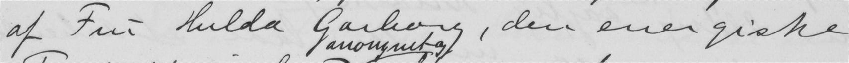af Fru Hulda Garborg, den energiske
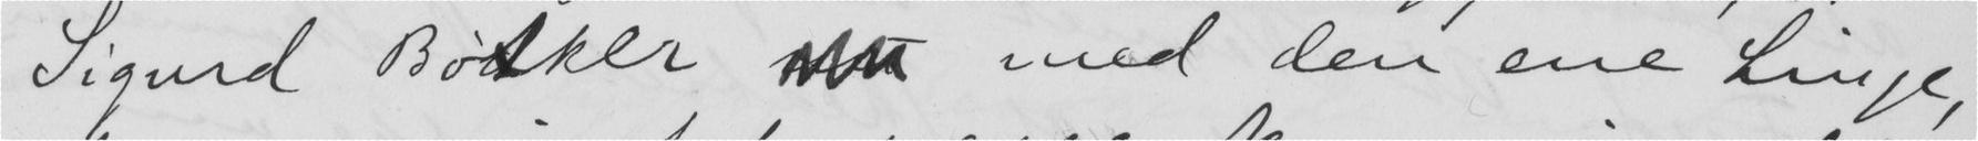Sigurd Bøtker  med den ene Linje,
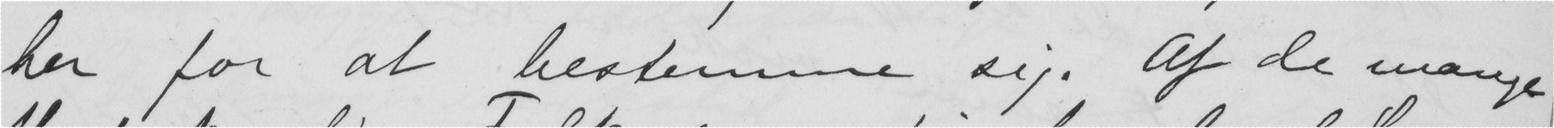her for at bestemme sig. Af de mange
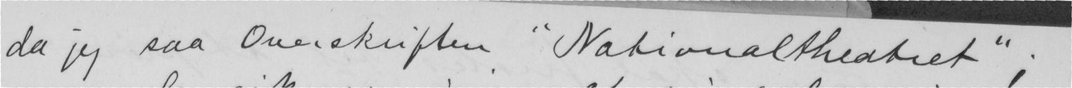da jeg saa Overskriften "Nationaltheatret";
